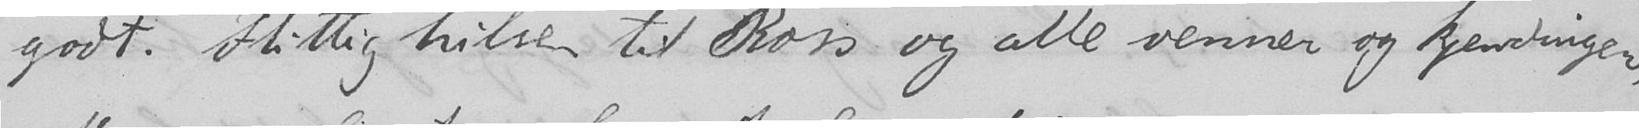godt. Flittig hilsen til Ross og alle venner og kjendinger,
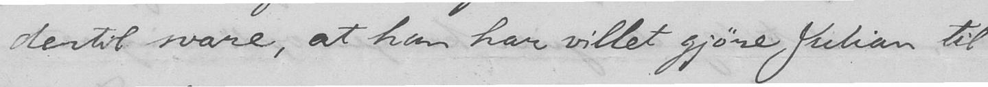dertil svare, at han har villet gjøre Julian til
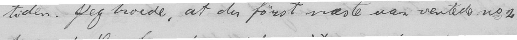tiden. Jeg troede, at du først næste aar ventede no 2
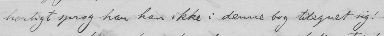herligt sprog har han ikke i denne bog tilegnet sig! -
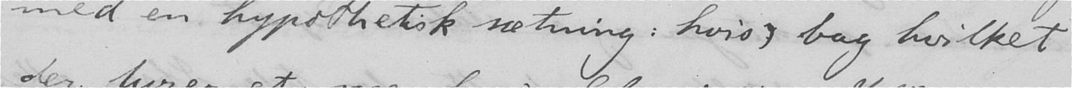med en hypothetisk sætning: hvis, bag hvilket
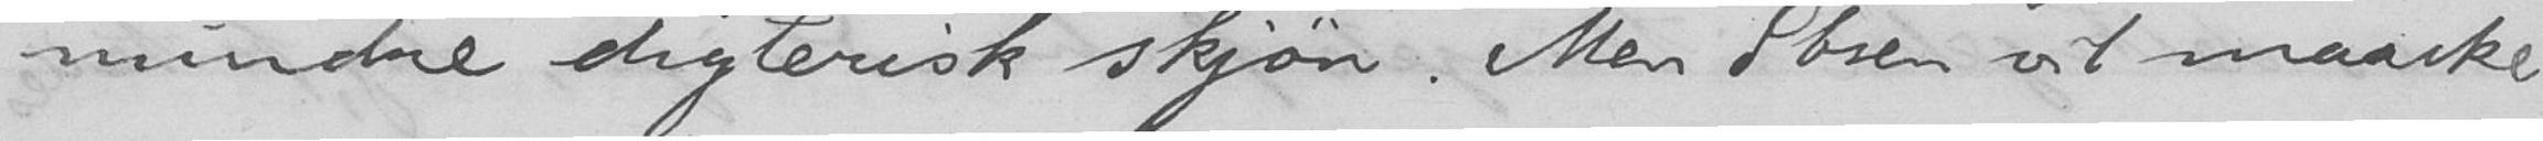mindre digterisk skjøn. Men Ibsen vil maaske
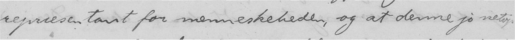repræsentant for menneskeheden, og at denne jo netop
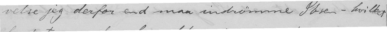velse jeg derfor end maa indrømme Ibsen - hvilket
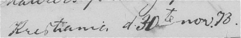Kristiania d. 30te nov. 73.
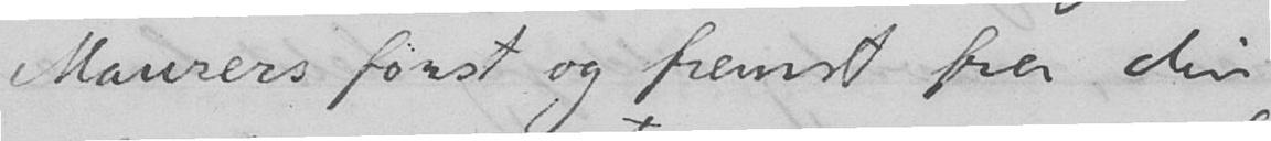Maurers først og fremst fra din
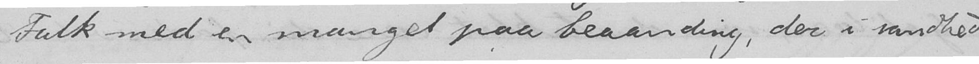Falk med en mangel paa beaanding, der i sandhed
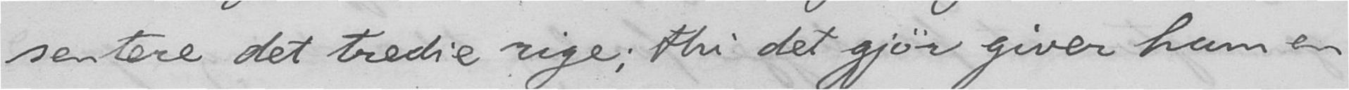sentere det tredie rige; thi det gjør giver ham en
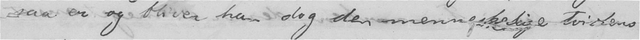saa er og bliver han dog den menneskelige tvivlens
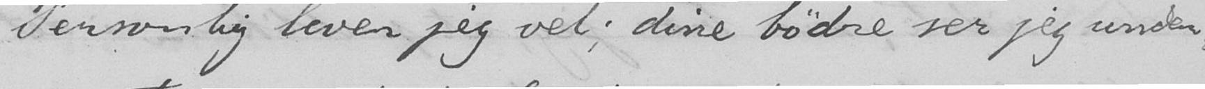Personlig lever jeg vel; dine bødre ser jeg under-
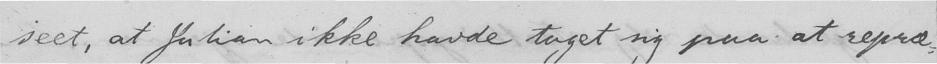seet, at Julian ikke havde taget sig paa at repræ-
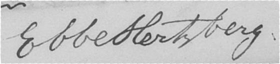Ebbe Hertzberg.
